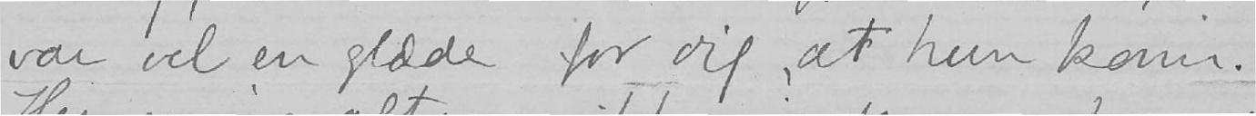var vel en glæde for dig, at hun kom.
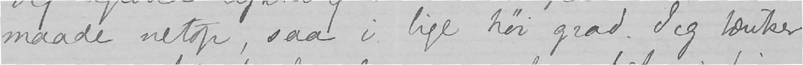maade netop, saa i lige høi grad. Jeg tænker
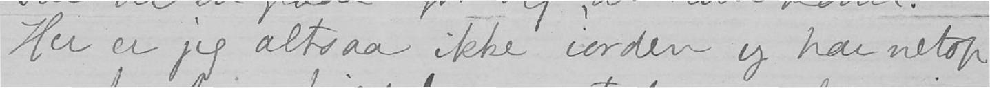Her er jeg altsaa ikke iorden og har netop
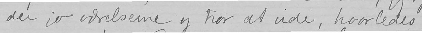der jo værelserne og tror at vide, hvorledes
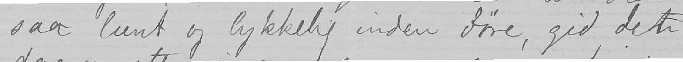saa lunt og lykkelig inden døre, gid den
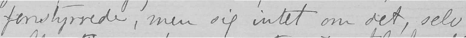forstyrrede, men sig intet om det, selv
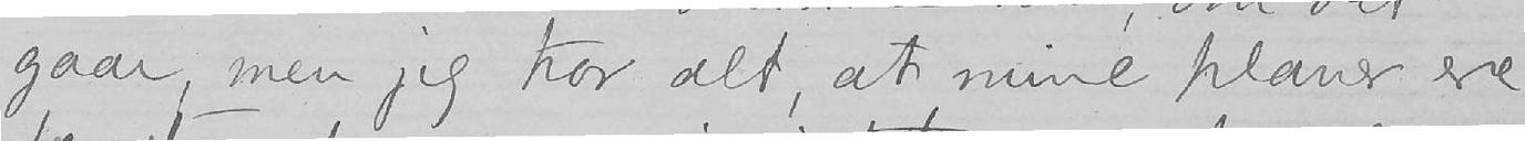gaar, men jeg tror alt, at mine planer ere
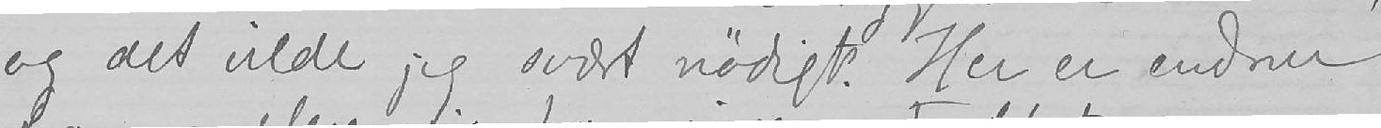og det vilde jeg svært nødigt. Her er endnu
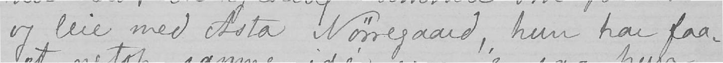og leie med Asta Nørregaard, hun har faa-
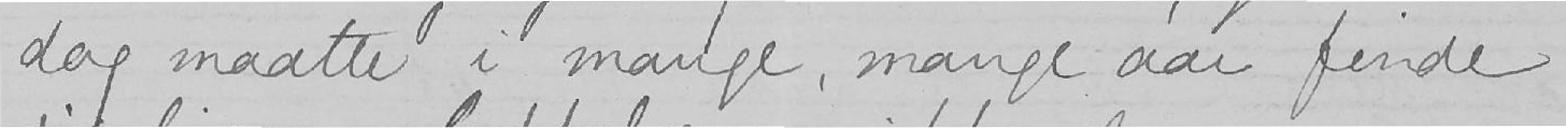dag maatte i mange, mange aar finde
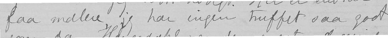faa malere, jeg har ingen truffet saa godt
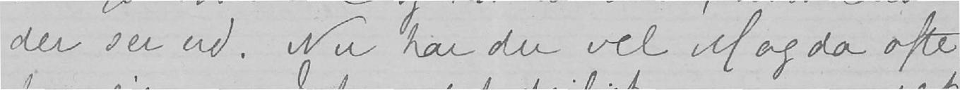der ser ud. Nu har du vel Magda ofte
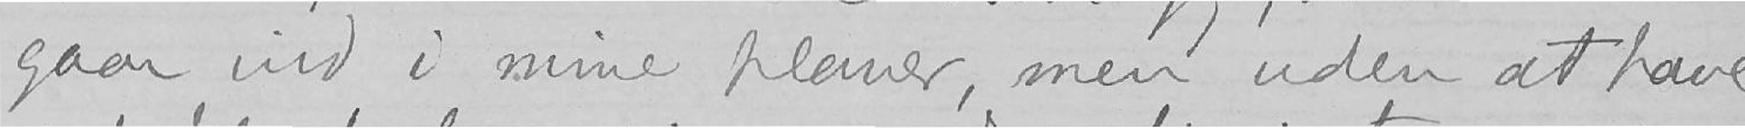gaar ind i mine planer, men uden at have
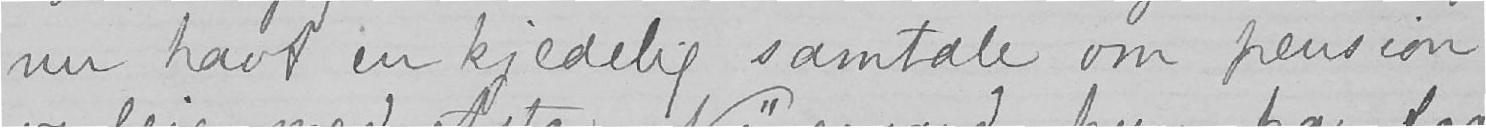nu havt en kjedelig samtale om pension
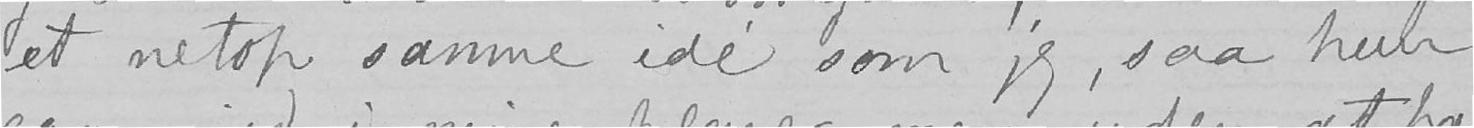et netop samme idé som jeg, saa hun
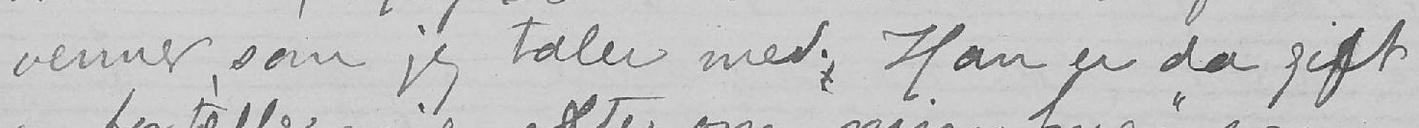venner, som jeg taler med. Han er da gift
